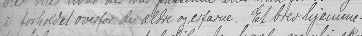i forholdet overfor de ældre og erfarne. Et brev hjemme-
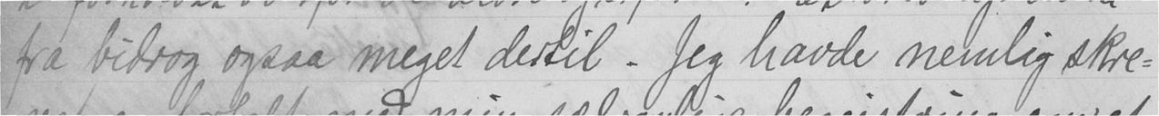fra bidrog ogsaa meget dertil. Jeg havde nemlig skre-
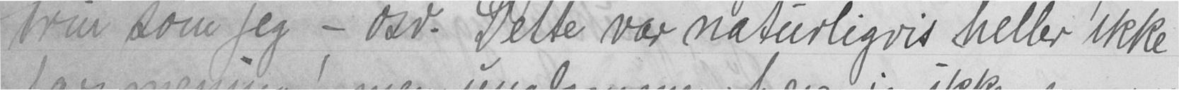trin som jeg -, osv. Dette var naturligvis heller ikke
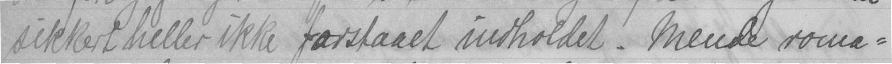sikkert heller ikke farstaaet indholdet. Mende roma-
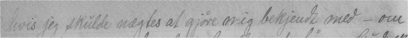hvis jeg skulde nægtes at gjøre mig bekjendt med - om
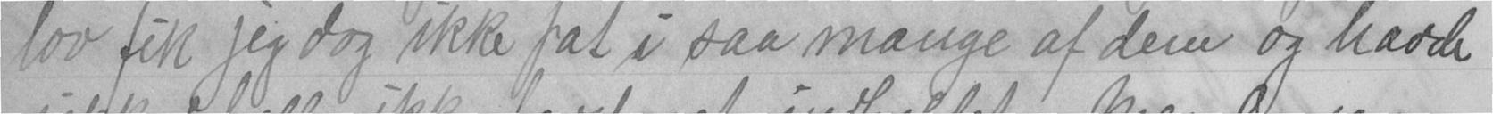lov fik jeg dog ikke fat i saa mange af dem og havde
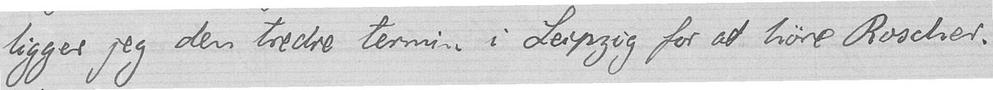ligger jeg den tredie termin i Leipzig for at høre Roscher.
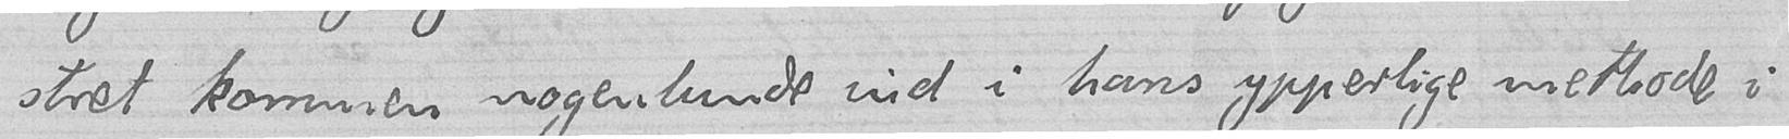stret kommen nogenlunde ind i hans ypperlige methode i
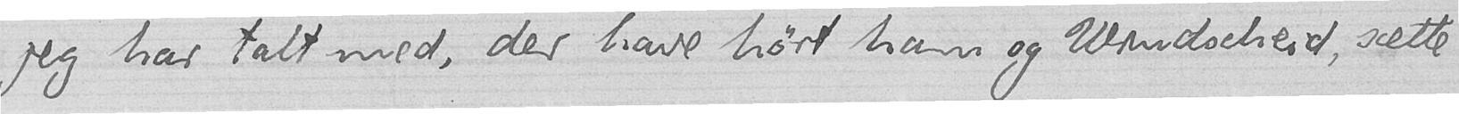jeg har talt med, der have hørt ham og Windscheid, sætte
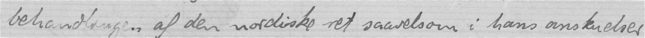behandlingen af den nordiske ret saavelsom i hans anskuelser
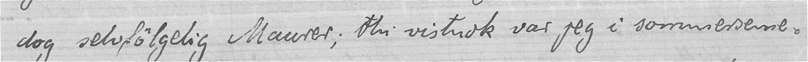dog selvfølgelig Maurer; thi vistnok var jeg i sommerseme-
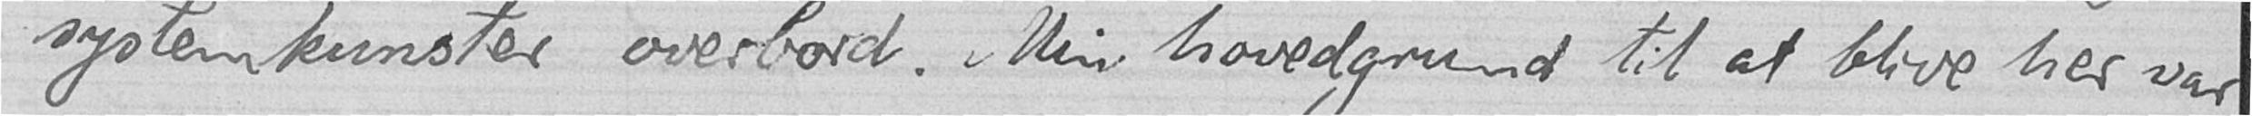systemkunster overbord. Min hovedgrund til at blive her var
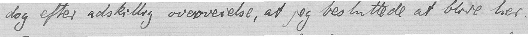dog efter adskillig overveielse, at jeg besluttede at blive her.
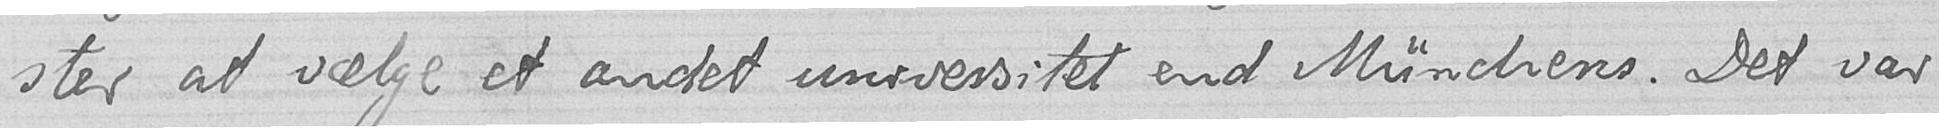ster at vælge et andet universitet end Münchens. Det var
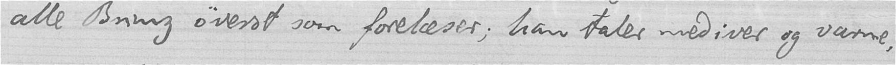alle Brinz øverst som forelæser; han taler med iver og varme,
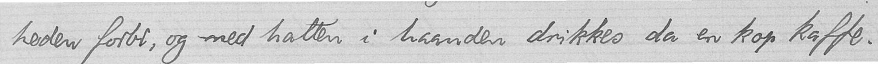heden forbi, og med hatten i haanden drikkes da en kop kaffe.
